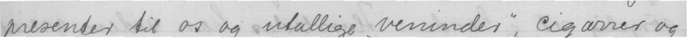presenter til os og utallige "veninder", cigarrer og
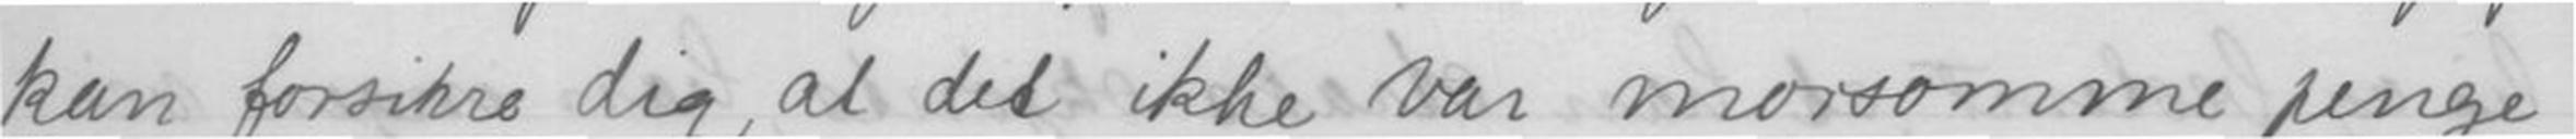kan forsikre dig, at det ikke var morsomme penge
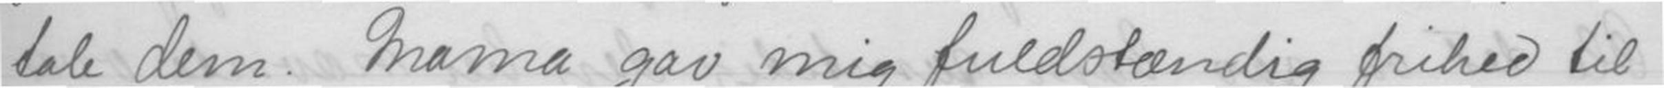tale dem. Mama gav mig fuldstændig frihed til
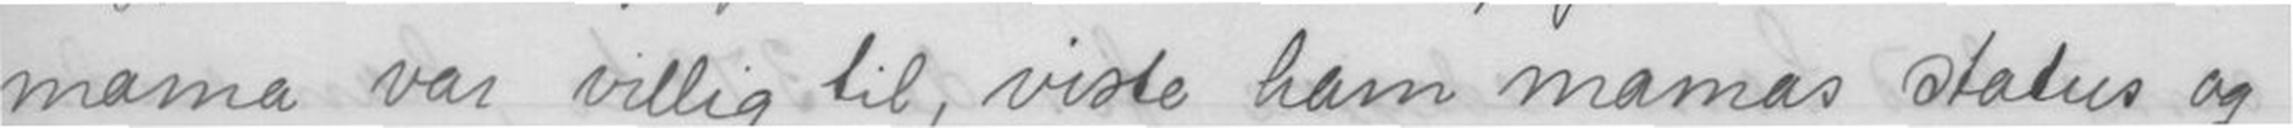mama var villig til, viste ham mamas status og
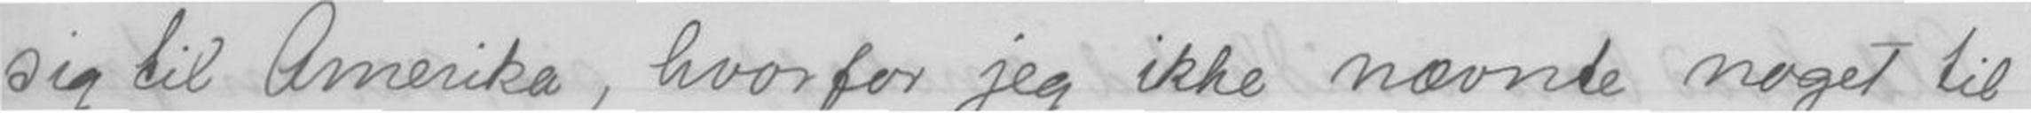sig til Amerika, hvorfor jeg ikke nævnte noget til
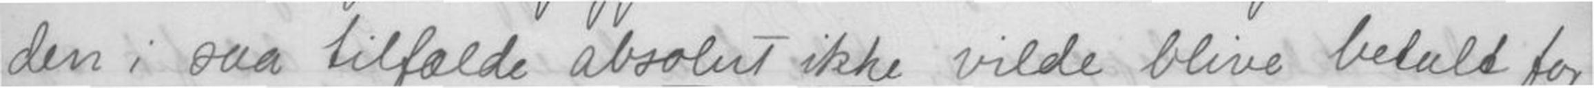den i saa tilfælde absolutut ikke vilde blive betalt for
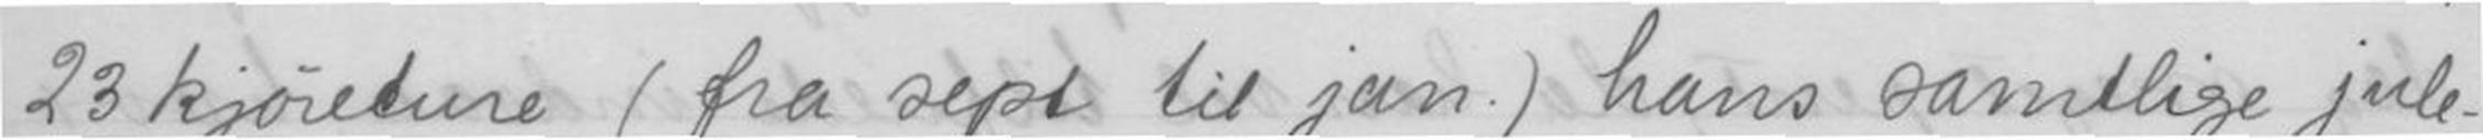23 kjøreture (fra sept til jan.) hans samtlige jule-
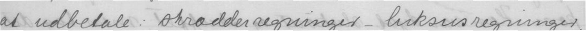at udbetale: skrædderregninger _ luksusregninger,
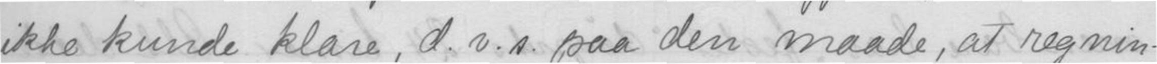ikke kunde klare, d.v.s. paa den maade, at regnin-
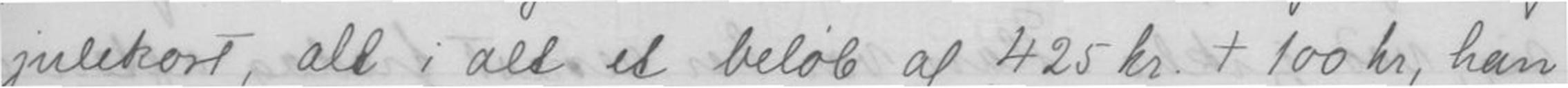julekort, alt i alt et beløb af 425 kr. + 100 kr, han
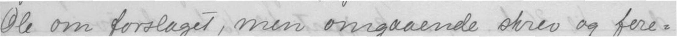Ole om forslaget, men omgaaende skrev og fore-
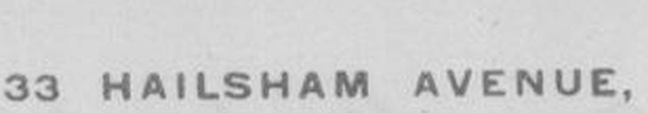33 HAILSHAM AVENUE,
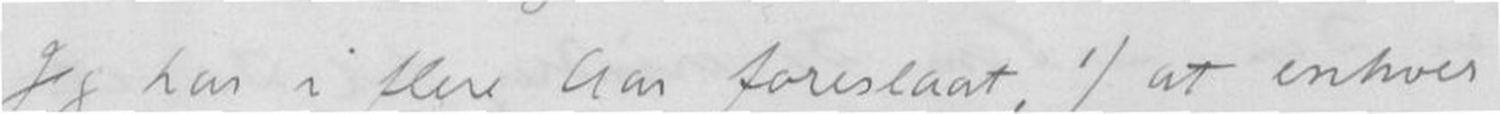Jeg har i flere Aar foreslaat, 1) at enhver
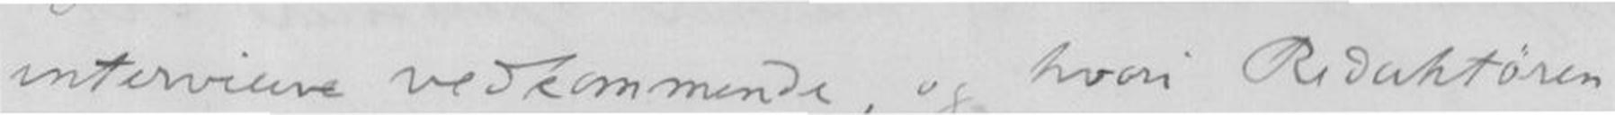interviewe vedkommende, og hvori Redaktøren
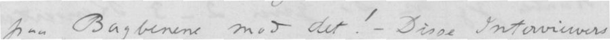paa Bagbenene mod det! - Disse Interviewers
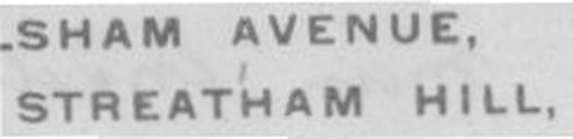STREATHAM HILL.
